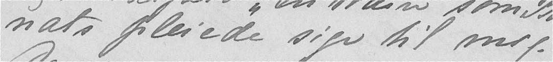nats pleiede sige til mig.
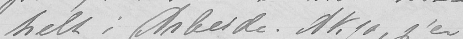helt i Arbeide. Ak ja, jeg er
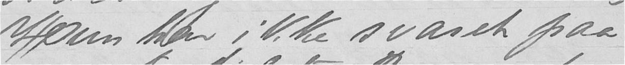Hun har ikke svaret paa
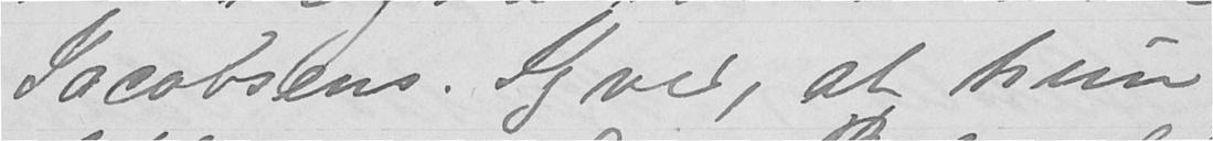Jacobsens. Jeg ved, at hun
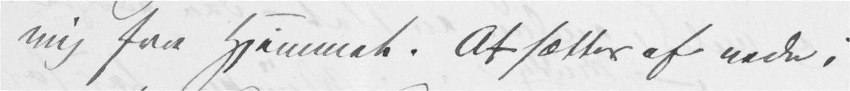mig fra Hjemmet. At sættes af nede i
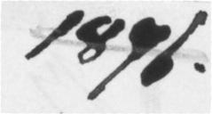1876.
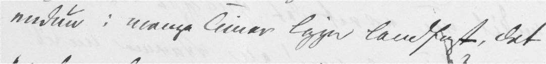endnu i mange Timer ligger landfast, det
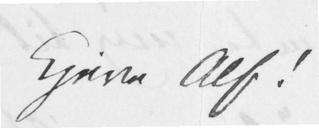Kjere Alf!
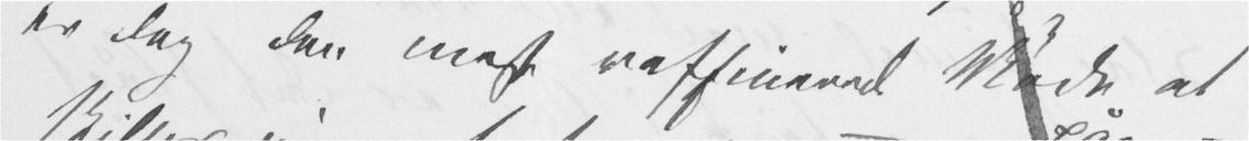er dog den mest raffinerede Måde at
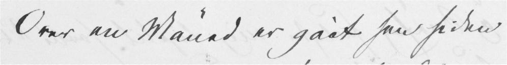Over en Måned er gået hen siden
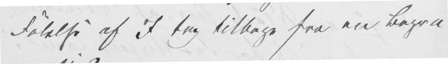Følelse af I tog tilbage fra en Begra-
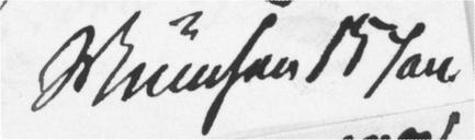München 15 Jan
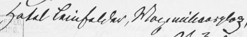Hotel Leinfelder Maximiliansplaz,
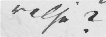velse?
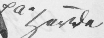Havde
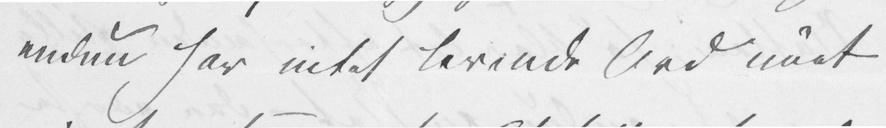endnu har intet levende Ord nået
